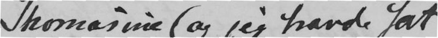Thomasine og jeg havde hat
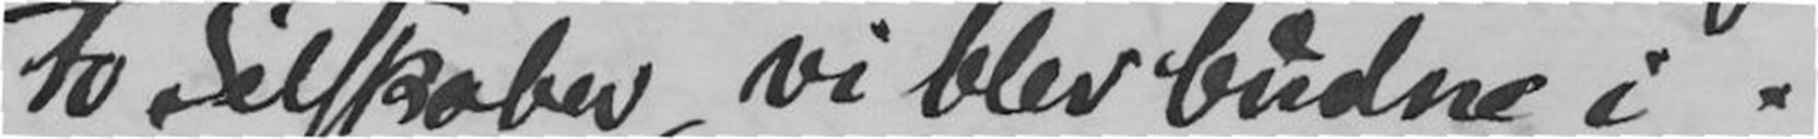to Selskaber, vi blev budne i.
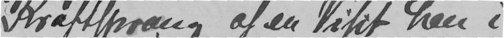Kraftsprang af en Visit hen i
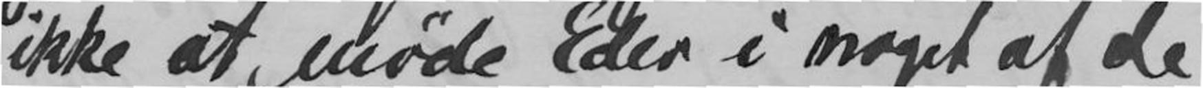ikke at møde Eder i noget af de
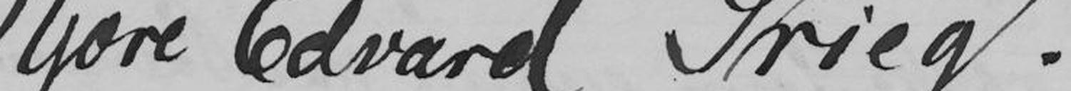Kjære Edvard Grieg!
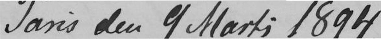Paris den 9 Marts 1894,
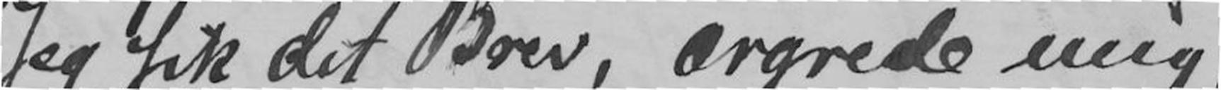Jeg fik dit Brev, ærgrede mig,
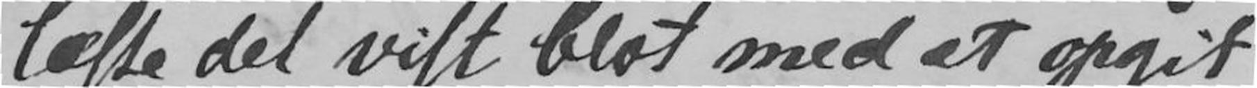læste det vist blot med et opgit
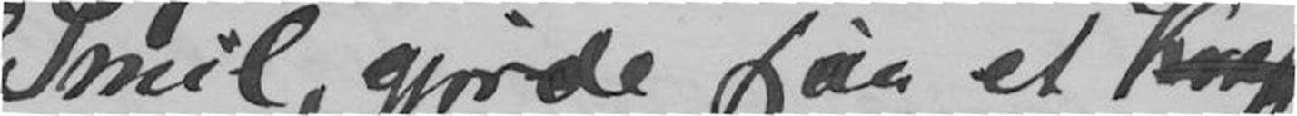Smil, gjorde saa et
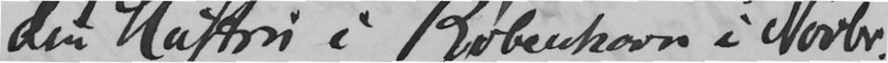din Hustru i Kjøbenhavn i Novbr.
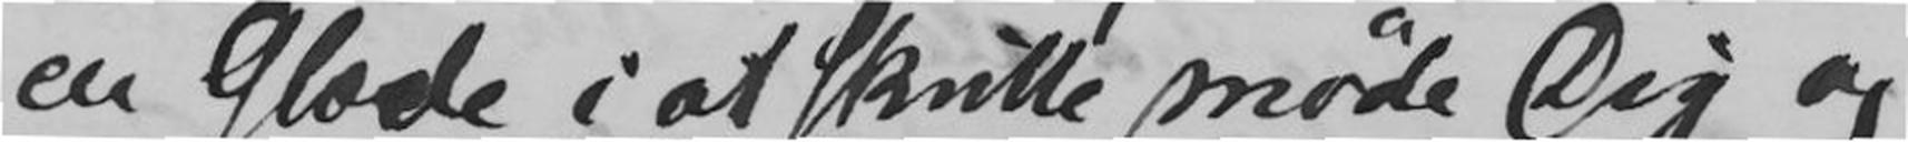en Glæde i at skulle møde Dig og
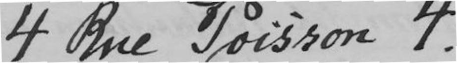4 Rue Poisson 4.
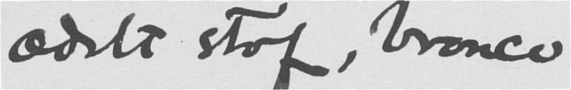ædelt stof, bronce
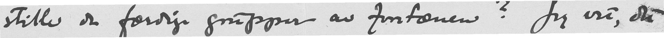stille de færdige grupper av fontænen? Jeg vet, det
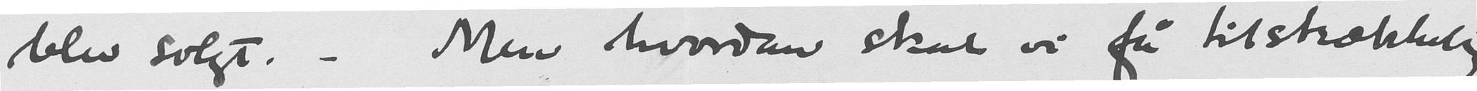blev solgt. - Men hvordan skal vi få tilstrækkelig
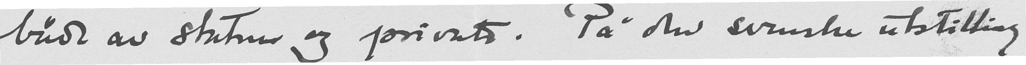både av staten og private. På den svenske utstilling
